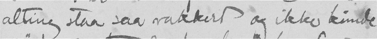alting staa saa vakkert og ikke kunde
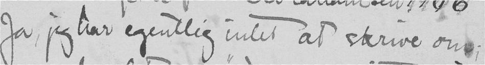Ja, jeg har egentlig intet at skrive om;
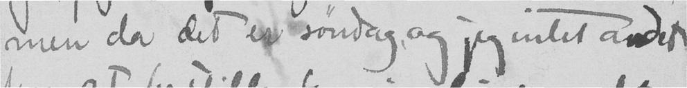men da det er søndag, og jeg intet andet
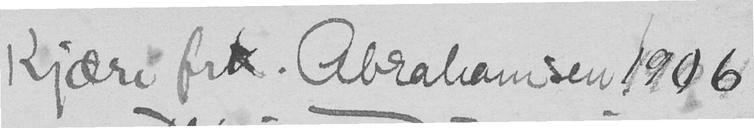Kjære frk. Abrahamsen 1906
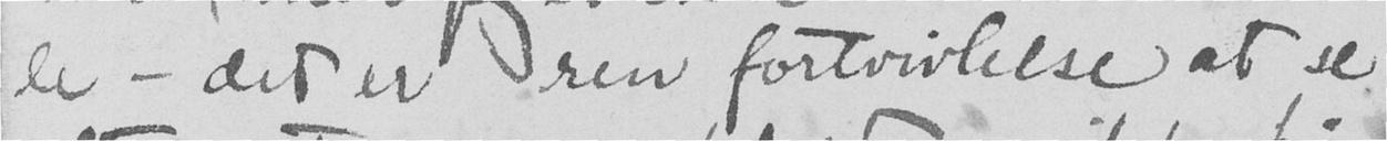le - det er ren fortvivlelse at se
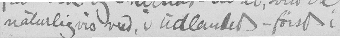naturligvis veed i udlandet - først i
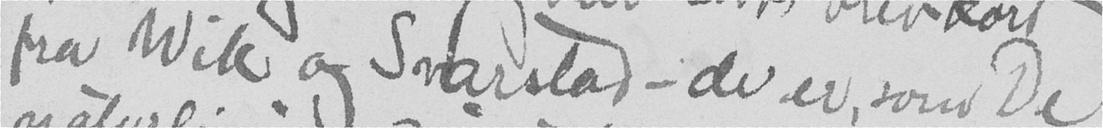fra Wik og Svarstad - de er, som De
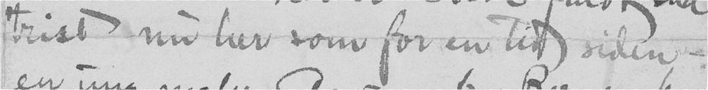trist nu her som for en tid siden-
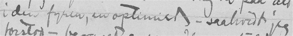i den fyren, en optemist - saahvidt jeg
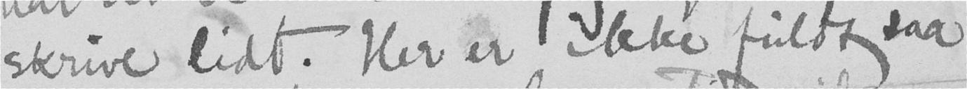skrive lidt. Her er ikke fuldt saa
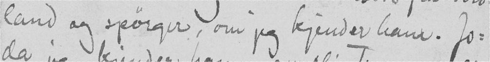land og spørger, om jeg kjender ham. Jo-
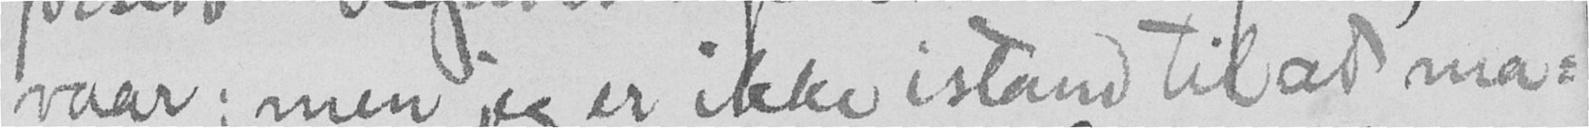vaar; men jeg er ikke istand til at ma-
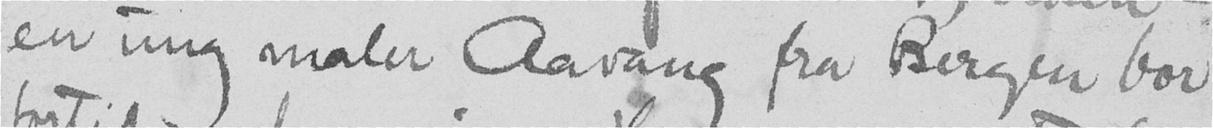en ung maler Aavang fra Bergen bor
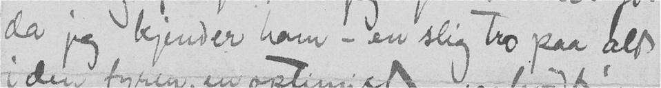da jeg kjender ham - en slig tro paa alt
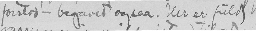forstod - begavet ogsaa. Her er fuld
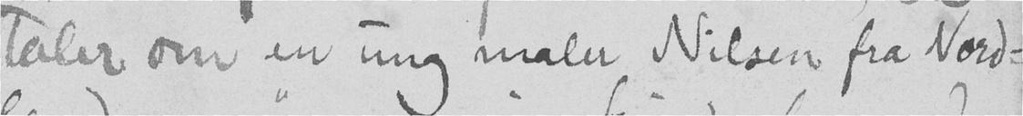taler om en ung maler Nilsen fra Nord-
